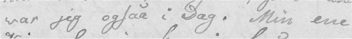var jeg ogsaa i Dag. Min ene
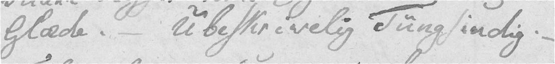Glæde. – Ubeskrivelig Tungsindig. –
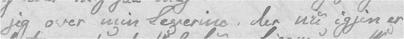jeg over min Severine, der nu igjen er
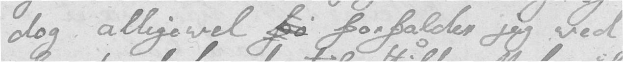dog alligevel fo forfalder jeg ved
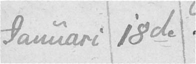Januari 18de
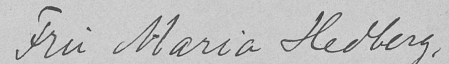Fru Maria Hedberg,
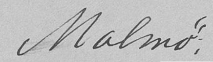Malmø.
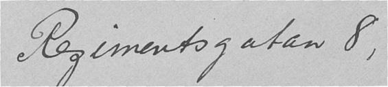Regimentsgatan 8,
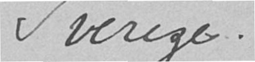Sverige.
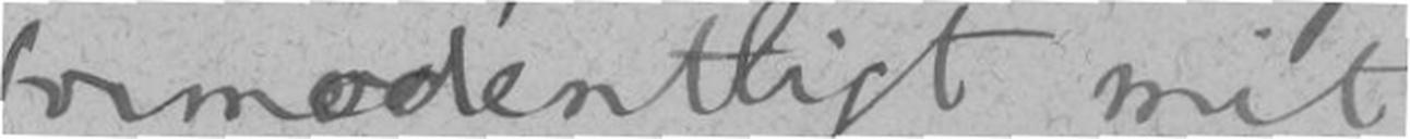formodentligt mit
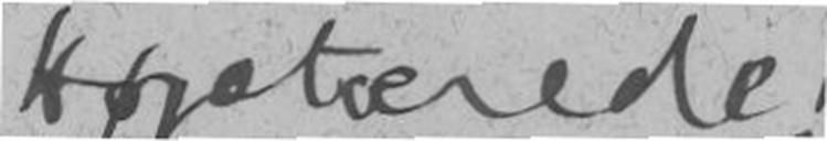Højstærede!
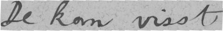De kan visst
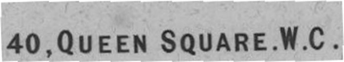40.QUEEN SQUARE.W.C.
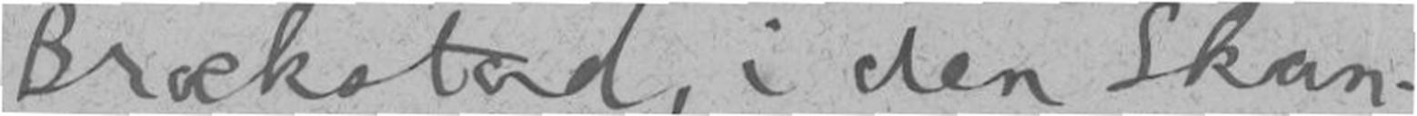Brækstad, i den Skan-
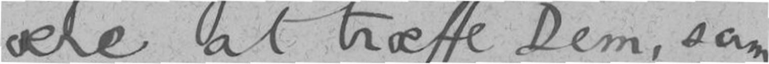ære at træffe Dem, sam-
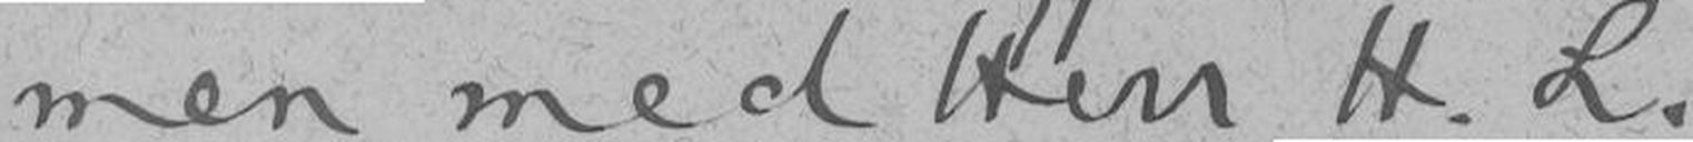men med Herr H.L.
